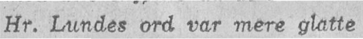Hr. Lundes ord var mere glatte
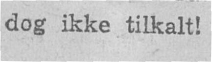dog ikke tilkalt!
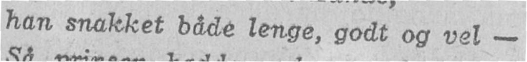han snakket både lenge, godt og vel -
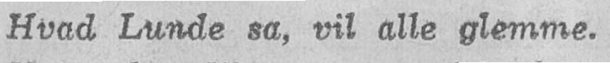Hvad Lunde sa, vil alle glemme.
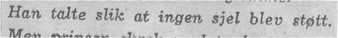Han talte slik at ingen sjel blev støtt.
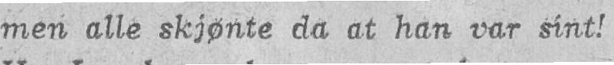men alle skjønte da at han var sint!
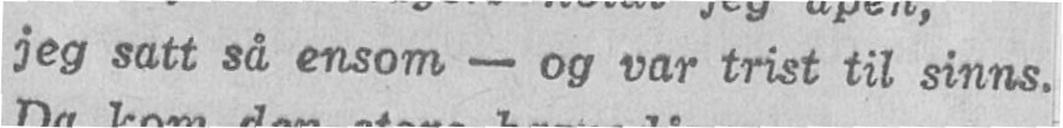jeg satt så ensom — og var trist til sinns.
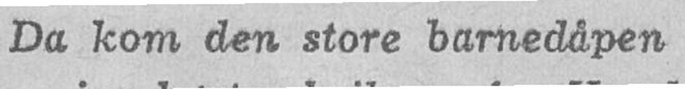Da kom den store barnedåpen
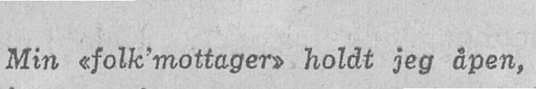Min "folk'mottager» holdt jeg âpen,
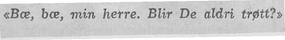«Bœ, bœ, min herre. Blir De aldri trøtt?»
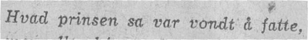Hvad prinsen sa var vondt å fatte,
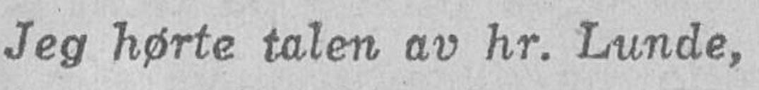Jeg hørte talen av hr. Lunde,
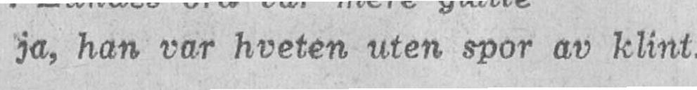- ja, han var hveten uten spor av klint.
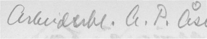Arbeiderbl. G. P. Åsen
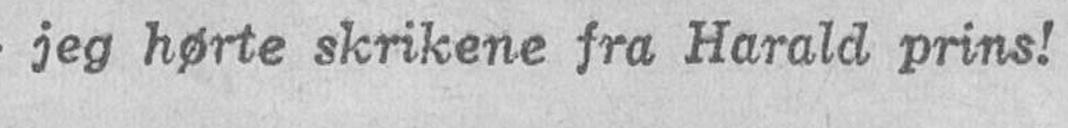- jeg hørte skrikene fra Harald prins!
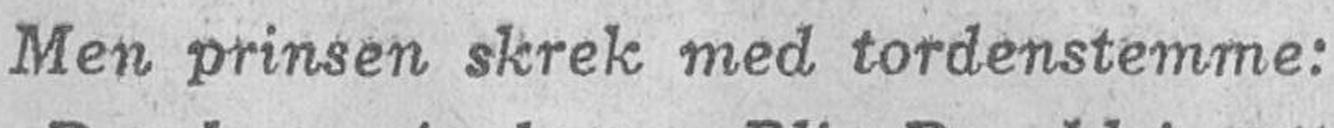Men prinsen skrek med tordenstemme:
